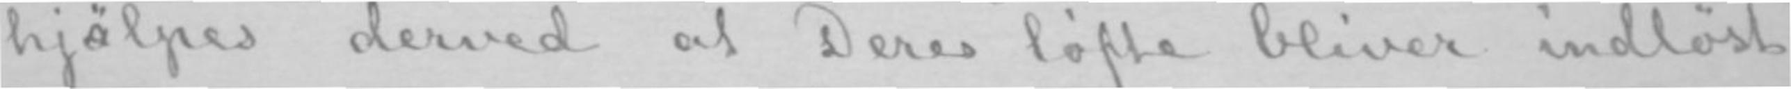hjälpes derved at Deres löfte bliver indlöst
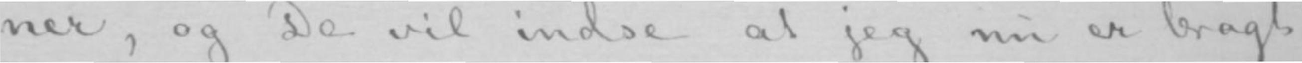ner, og De vil indse at jeg nu er bragt
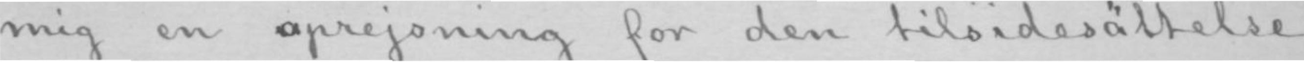mig en oprejsning for den tilsidesättelse
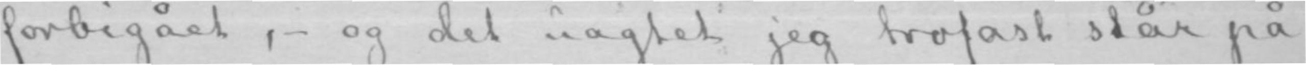forbigået,- og det uagtet jeg trofast står på
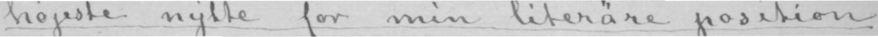höjeste nytte for min literäre position
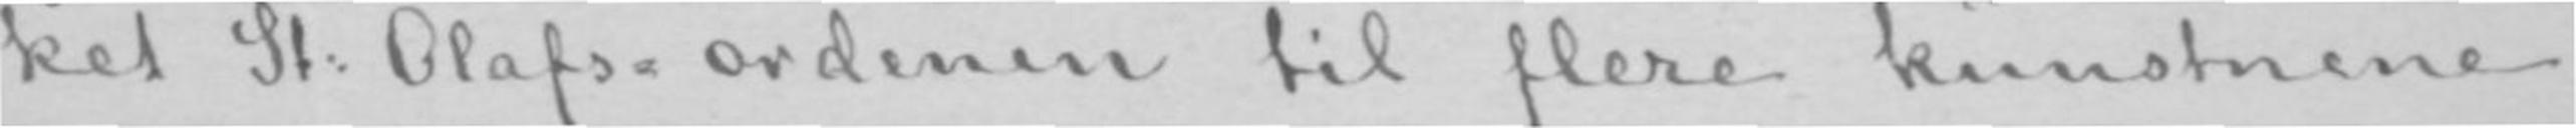ket St: Olafs-ordenen til flere kunstnere,
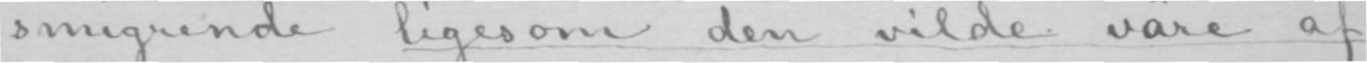smigrende ligesom den vilde väre af
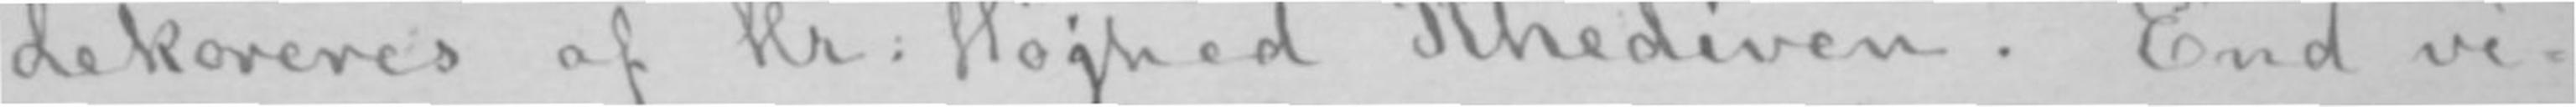dekoreres af Hr: Höjhed Khediven. End vi-
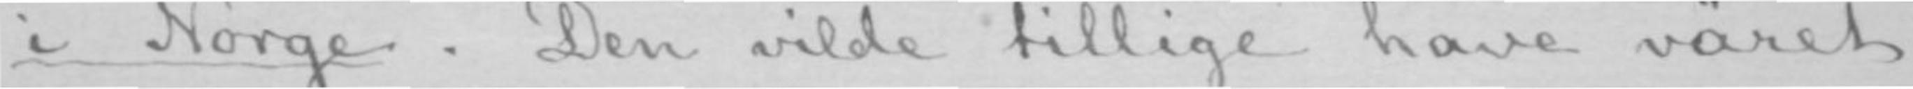i Norge. Den vilde tillige have väret
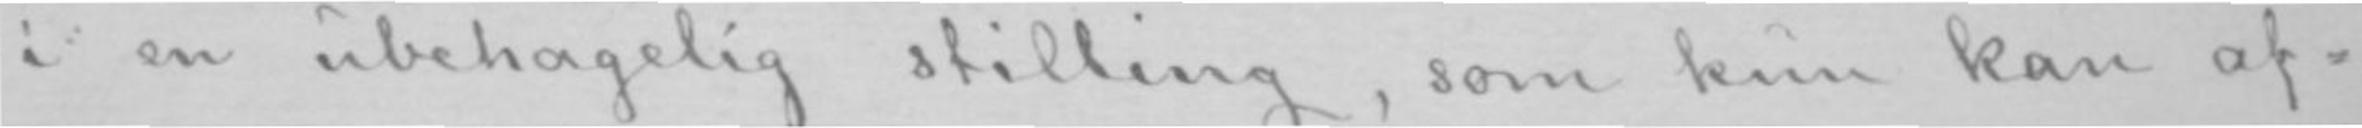i en ubehagelig stilling, som kun kan af-
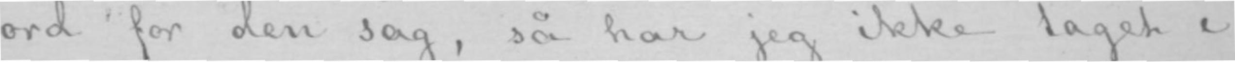ord for den sag, så har jeg ikke taget i
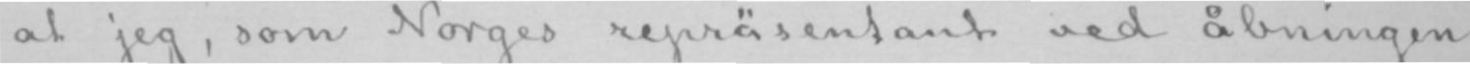at jeg, som Norges repräsentant ved åbningen
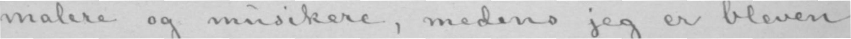malere og musikere, medens jeg er bleven
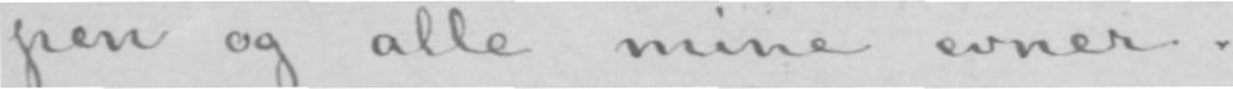pen og alle mine evner.
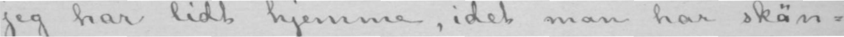jeg har lidt hjemme, idet man har skän-
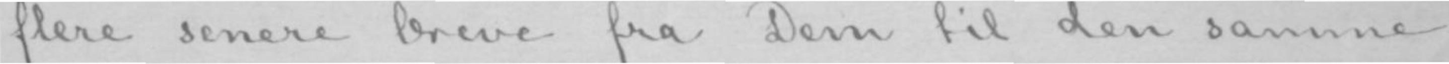flere senere breve fra Dem til den samme
